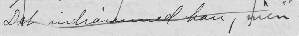Det indrømmed han, men -
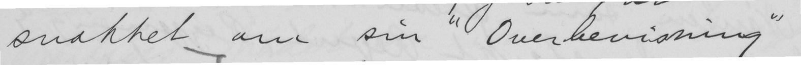snakket om sin "Overbevisning"
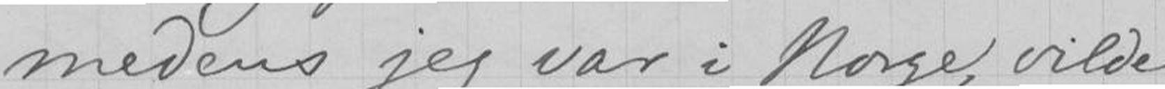medens jeg var i Norge, vilde
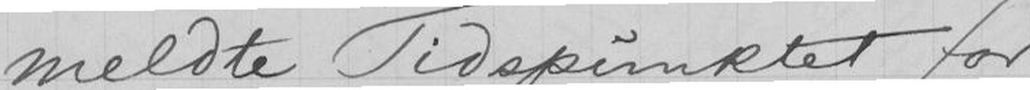meldte Tidspunktet for
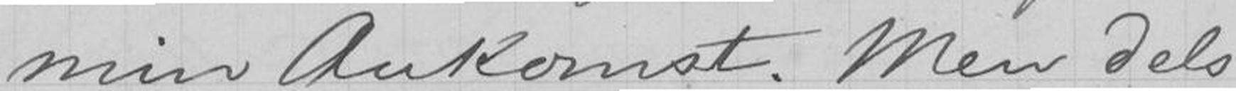min Ankomst. Men dels
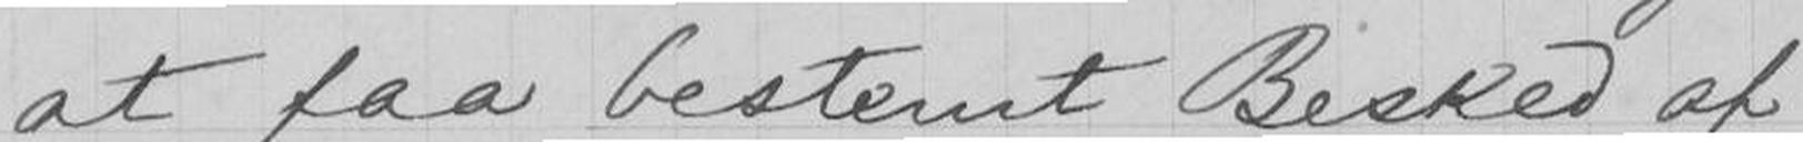at faa bestemt Besked af
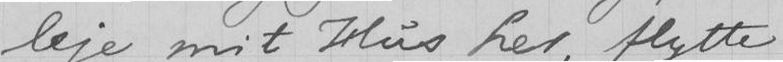leje mit Hus her, flytte ud
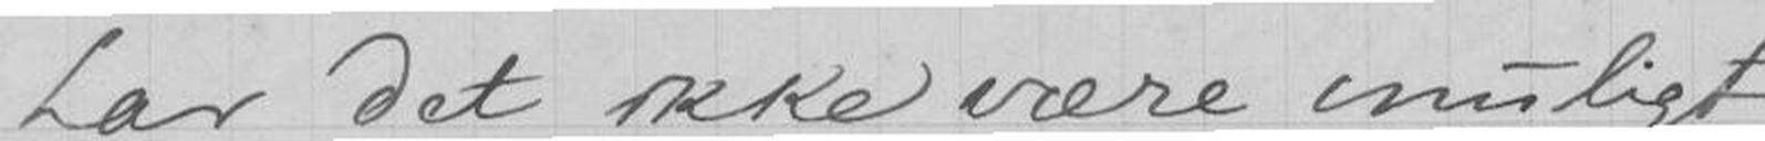har det ikke være muligt
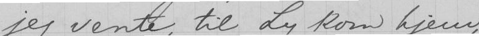jeg vente, til Ly kom hjem,
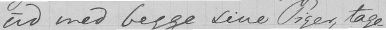med begge sine Piger, tage
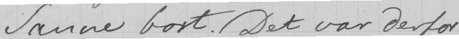Sanne bort. Det var derfor
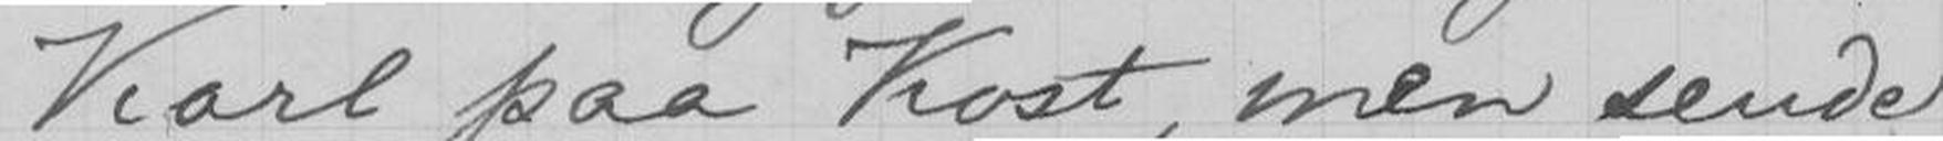Karl paa Kost, men sende
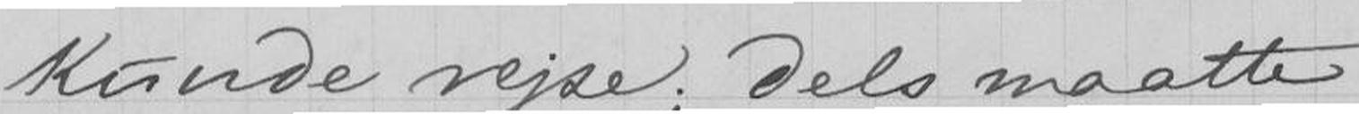kunde rejse; dels maatte
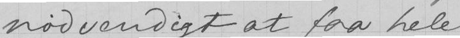nødvendigt at faa hele
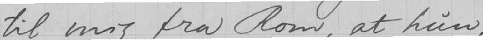til mig fra Rom, at hun,
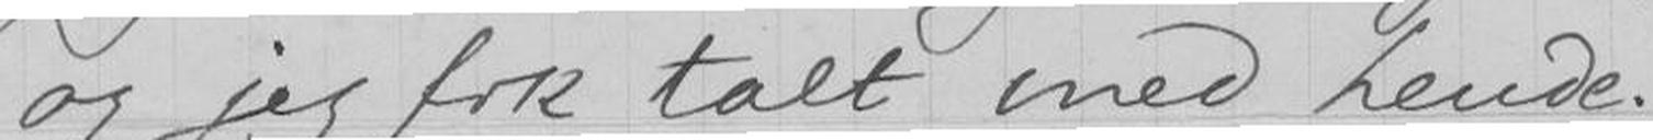og jeg fik talt med hende.
